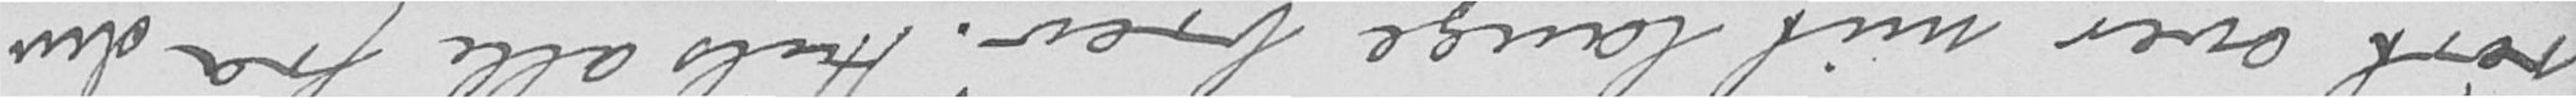rørt over mit lange brev. Hils alle fra din
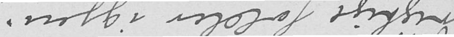rigtige folder igjen.
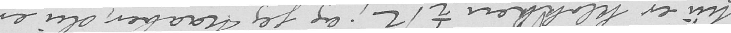Nu er klokken 1/2 12; og jeg haaber, du er
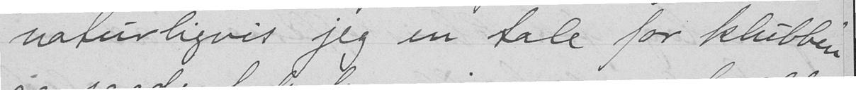naturligvis jeg en tale for klubben
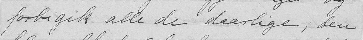forbigik alle de daarlige; den
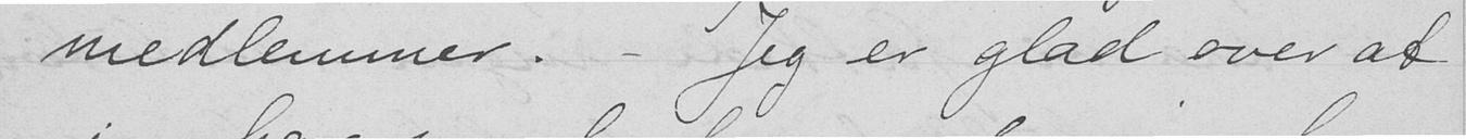medlemmer. - Jeg er glad over at
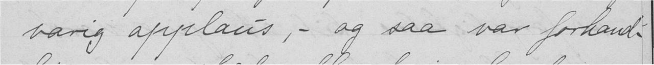varig applaus, - og saa var forhand-
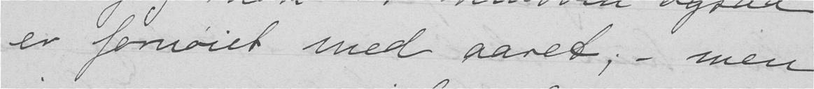er fornøiet med aaret, - men
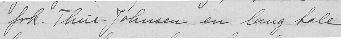frk. Thue-Johnsen en lang tale
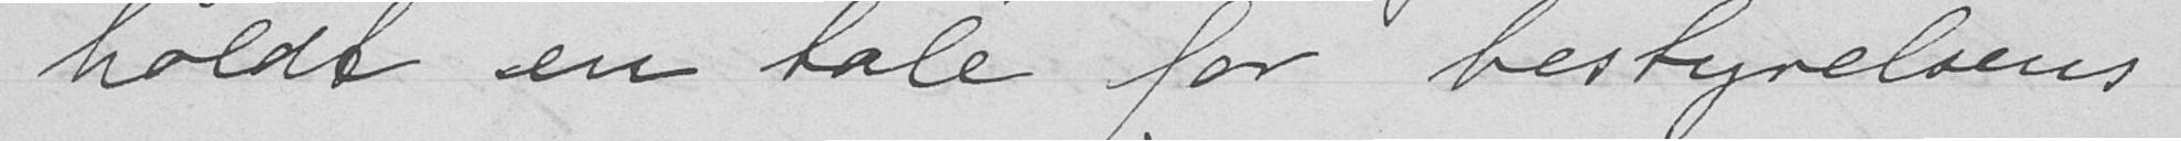holdt en tale for bestyrelsens
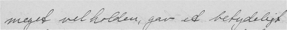meget velholden, gav et betydeligt
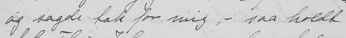og sagde tak for mig, - saa holdt
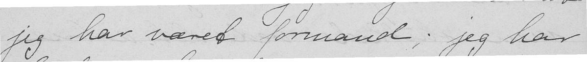jeg har været formand; jeg har
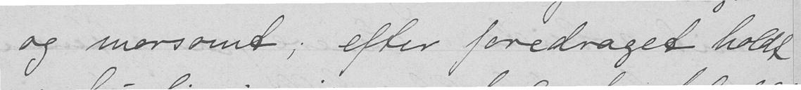og morsomt; efter foredraget holdt
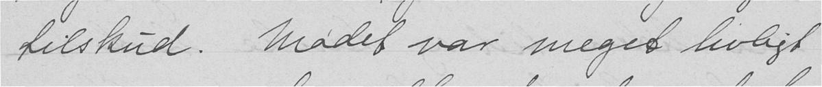tilskud. Mødet var meget livligt
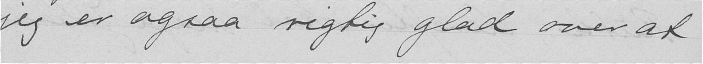jeg er ogsaa rigtig glad over at
